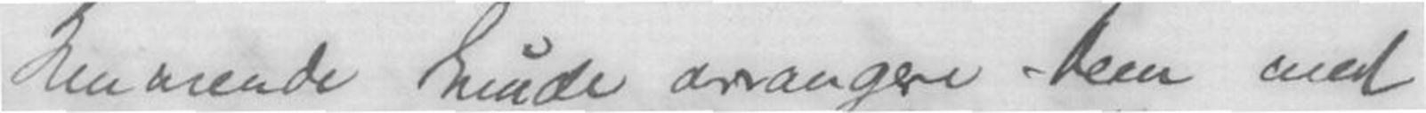kommende kunde arrangere Dem med
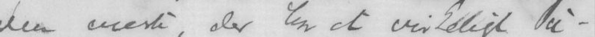den eneste, der har et virkeligt Pu-
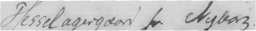Hesselagergaard pr Nyborg
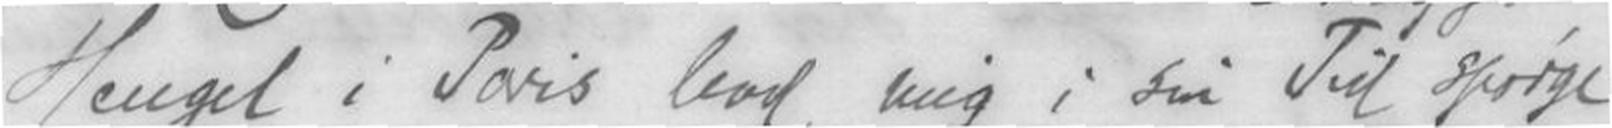Heugel i Paris bad mig i sin Tid spørge
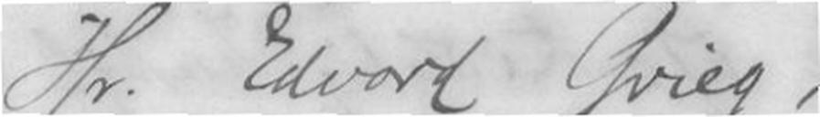Hr. Edvard Grieg
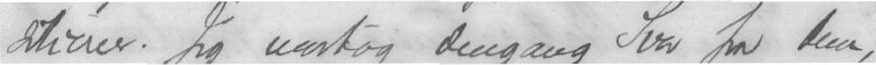sitioner. Jeg modtog dengang Svar fra Dem,
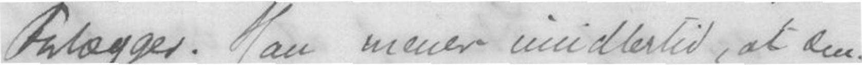Forlægger. Han mener imidlertid, at den-
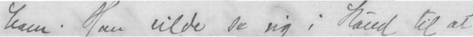ham. Han vilde se sig i stand til at
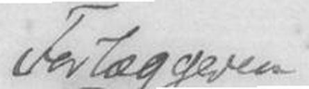Forlæggeren
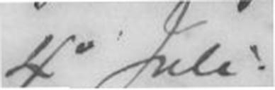4 juli
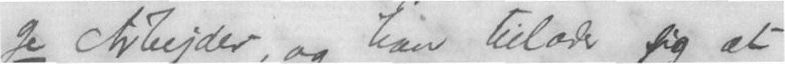ge Arbeider, og kan tillade sig at
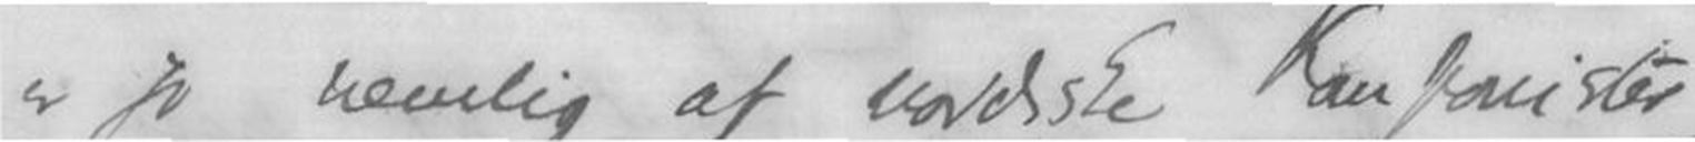er jo nemlig af nordisk Komponister
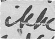ikke
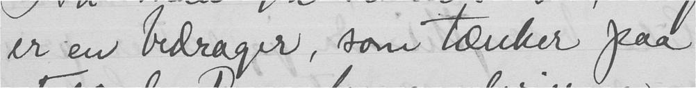er en bedrager, som tænker paa
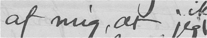af mig, at jeg
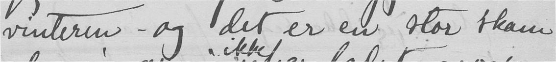vinteren - og det er en stor skam
